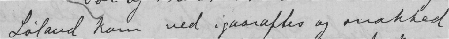Løland kom ved igaaraftes og snakked
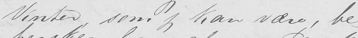Vinter, som jeg kan være, be-
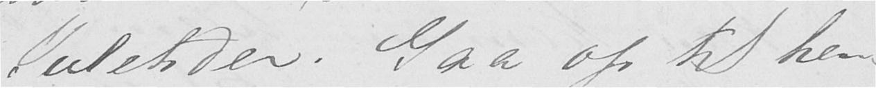Juletider. Gaa op til hen-
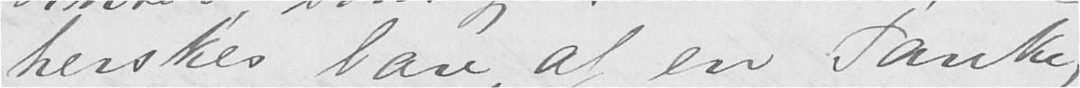herskes bare af en Tanke,
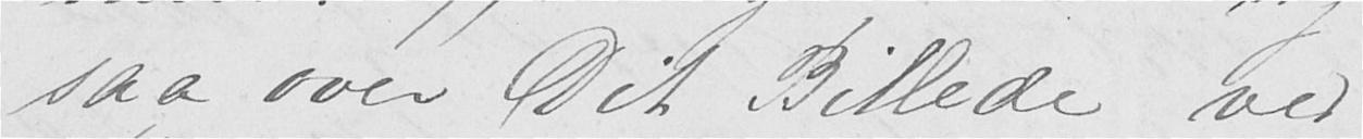saa over Dit Billede ved
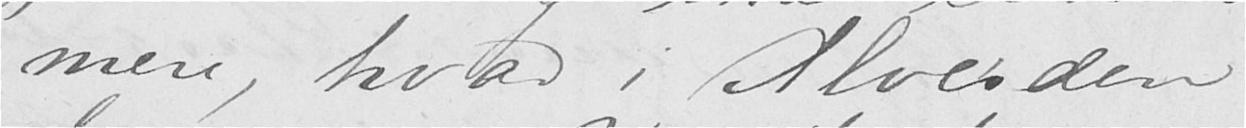mere, hvad i Alverden
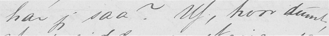har jeg saa? Uf, hvor dumt
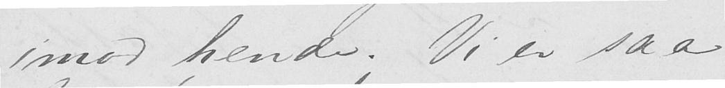imod hende. Vi er saa
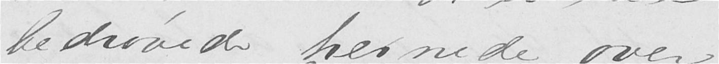bedrøvede hernede over
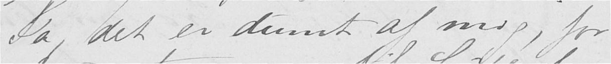Ja, det er dumt af mig, for
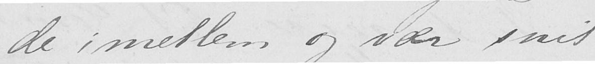de i mellem og vær snil
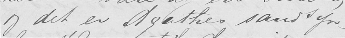og det er Agathes sandsyn-
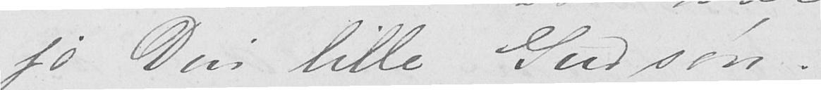jo Din lille Gudsøn.
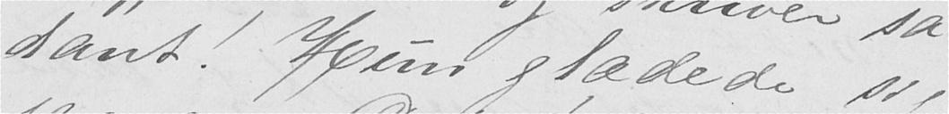dant. Hun glædede sig
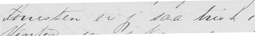Forresten er jeg saa trist i
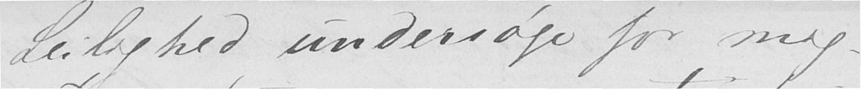Leilighed, undersøge for mig.
